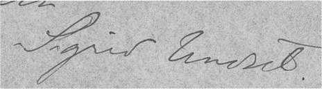Sigrid Undset.
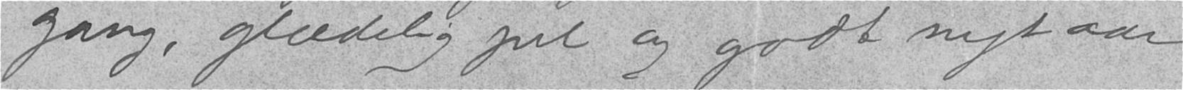gang, glædelig jul og godt nytaar!
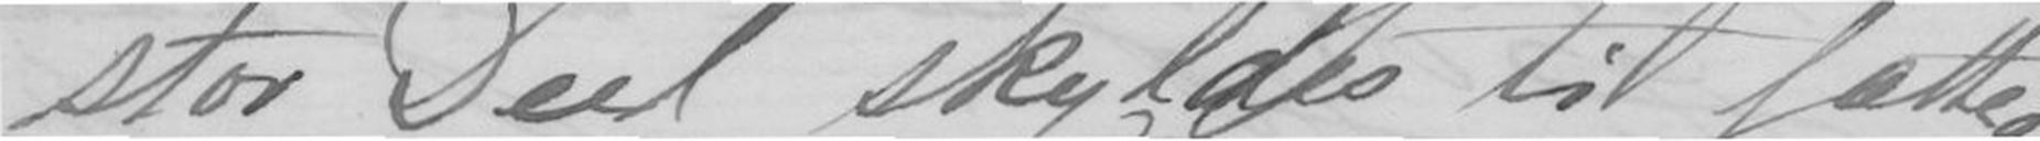stor Deel skyldes til fattige
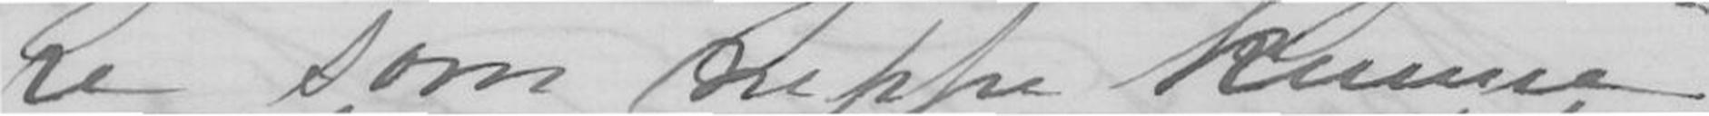re som neppe kunne
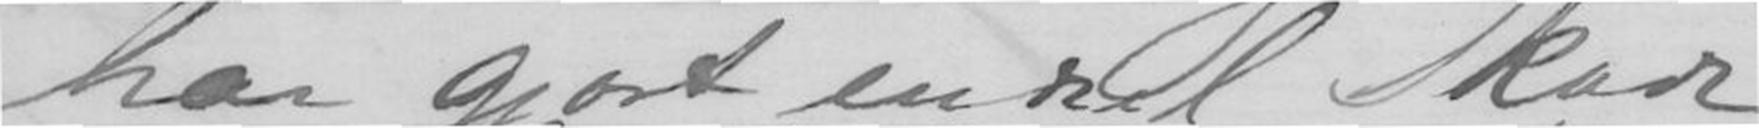har gjort endel Skade
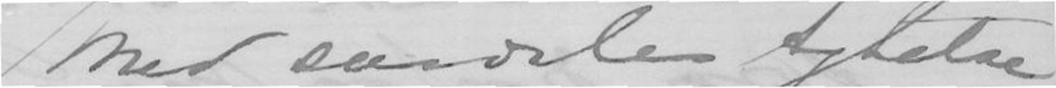Med sandeles Agtelse
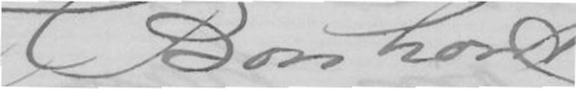F Borchorst
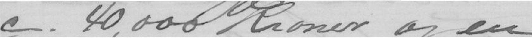c. 40,000 Kroner og en
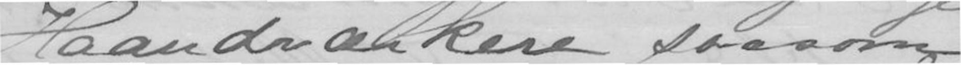Haandværkere saasom
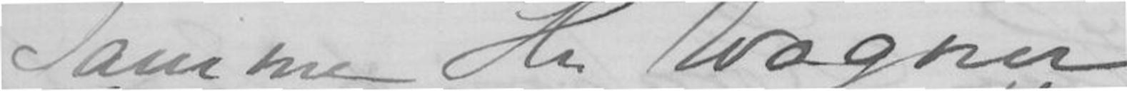Samme Hr Wagner
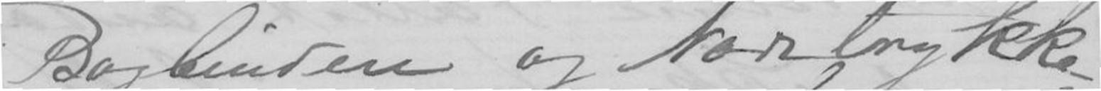Bogbindere og Nodetrykke-
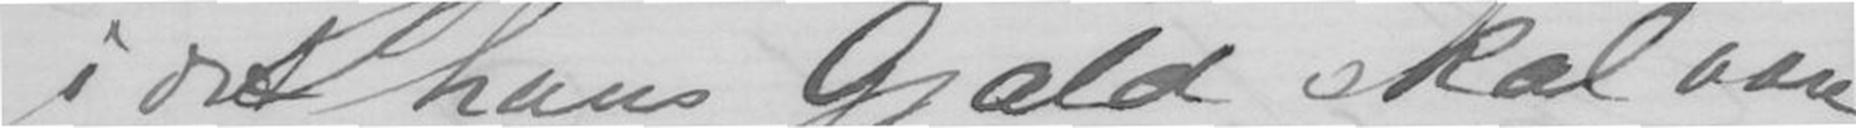i det hans Gjæld skal være
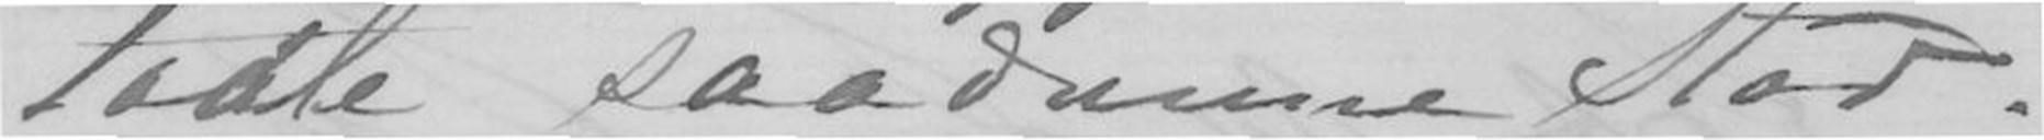taale saadanne Stød.
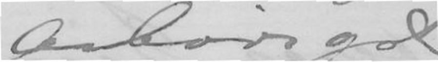Ærbødigst
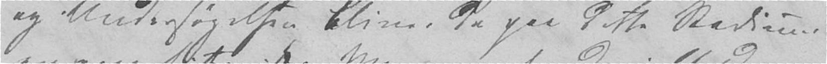og Undersøgelsen bliver da paa dette Stadium
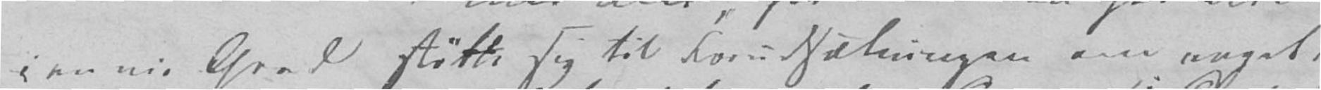i en vis Grad støtte sig til Forudsætningen om noget
